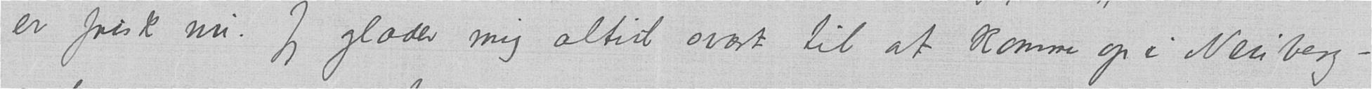er frisk nu. Jeg glæder mig altid svært til at komme op i Neuberg-
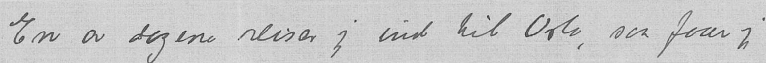En av dagene reiser jeg ind til Oslo, saa faar jeg
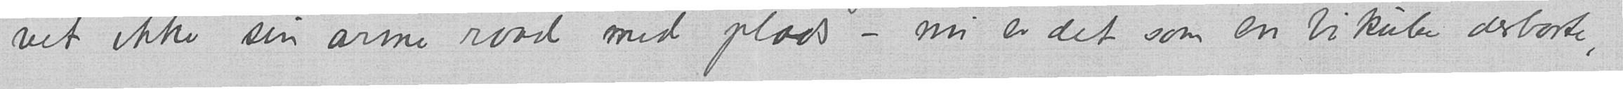vet ikke sin arme raad med plads – nu er det som en bikube derborte,
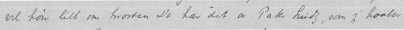vel høre litt om hvordan De har det av Pater Ludz, som jeg haaber
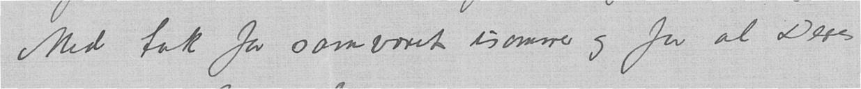Med tak for samværet isommer og for al Deres
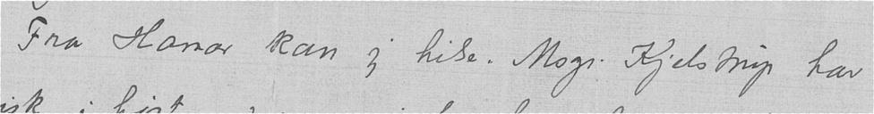Fra Hamar kan jeg hilse. Msgr. Kjelstrup har
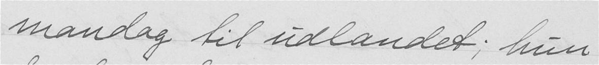mandag til udlandet; hun
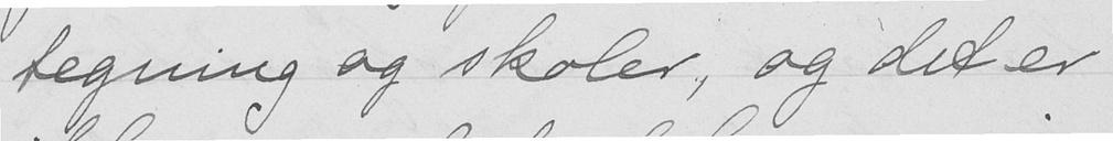tegning og skoler, og det er
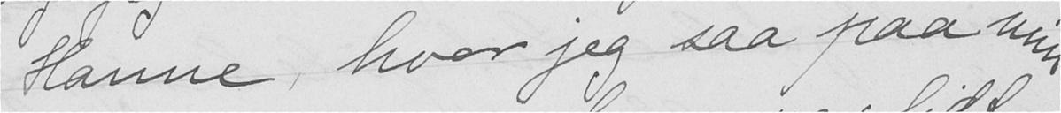Hanne, hvor jeg saa paa min
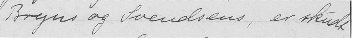Bryns og Svendsens, er skudt
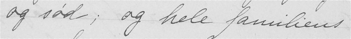og sød; og hele famliens
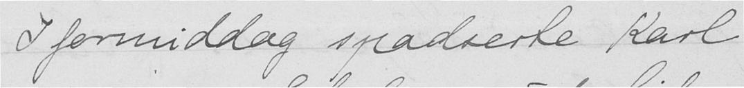I formiddag spadserte Karl
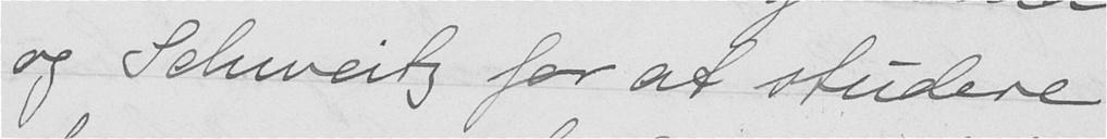og Schweitz for at studere
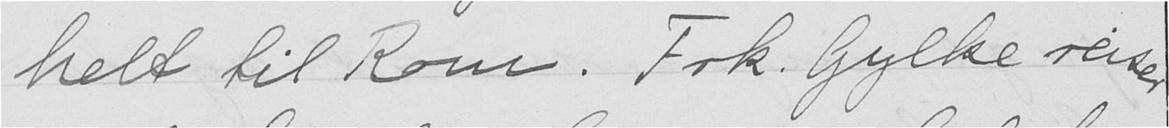helt til Rom. Frk. Gylke reiser
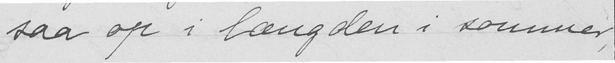saa op i længden i sommer
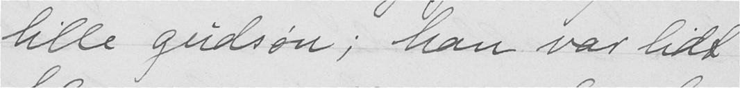lille gudsøn; han var lidt
